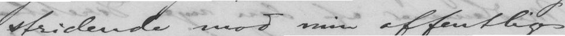stridende mod min offentlige
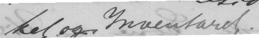ket og Inventaret.
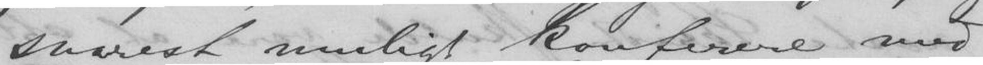snarest muligt konferere med
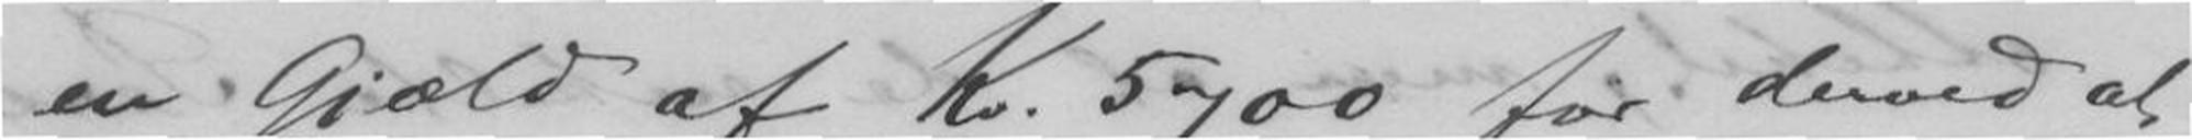en Gjæld af Kr. 5700 for derved at
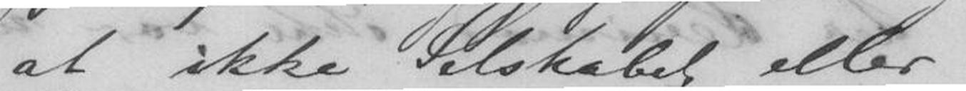at ikke Selskabet eller
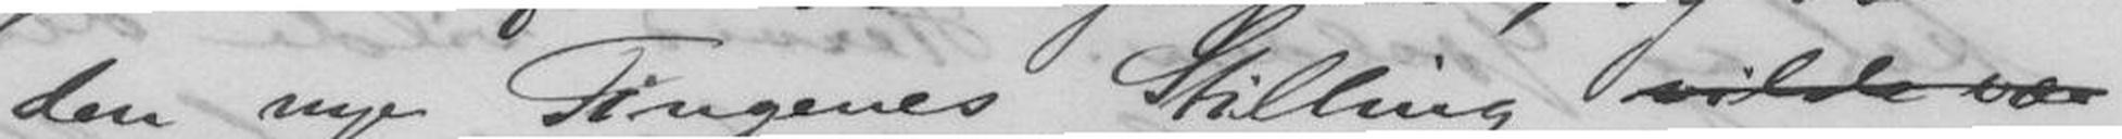den nye Tingenes Stilling vilde vær
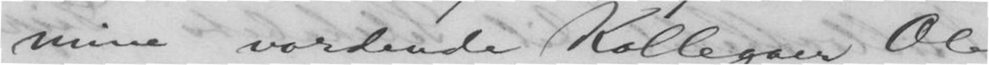mine vordende Kollegaer Ole
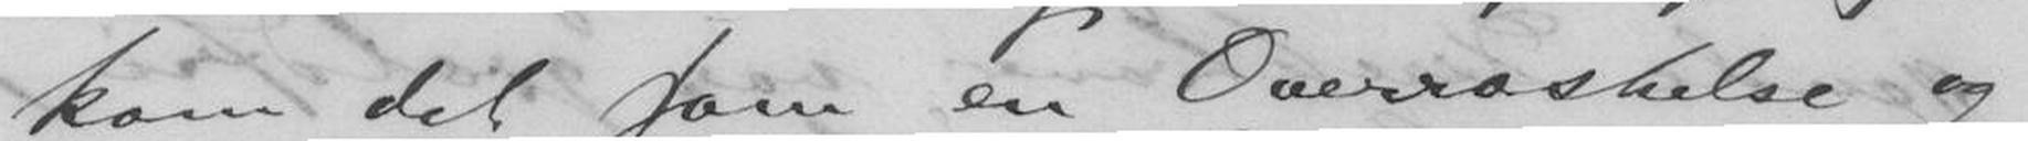kom det som en Overraskelse og-
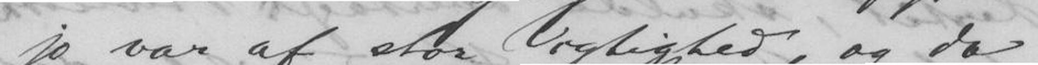jo var af stor Vigtighed, og da
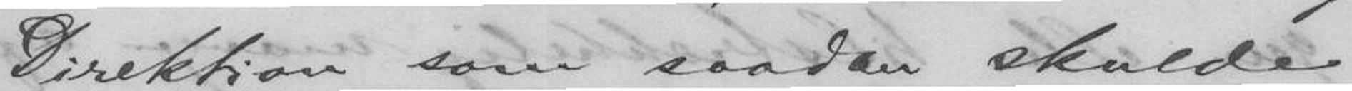Direktion som saadan skulde
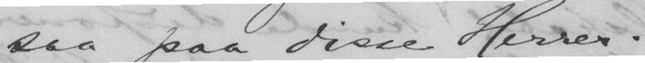saa paa disse Herrer.
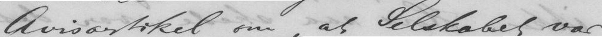Avisartikel om, at Selskabet var
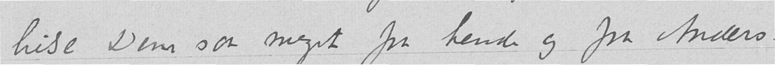hilse Dem saa meget fra hende og fra Anders.
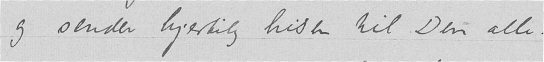og sender hjertelig hilsen til Dem alle.
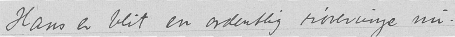Hans er blit en ordentlig røverunge nu.
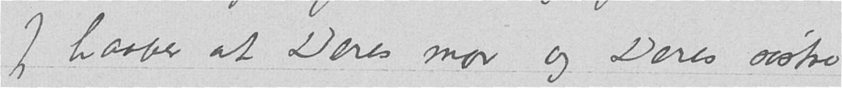Jeg haaber at Deres mor og Deres søstre
